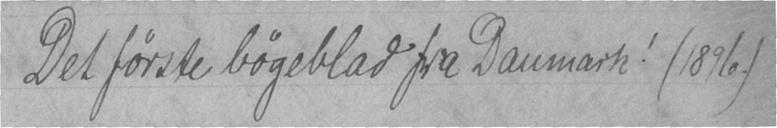Det første bøgeblad fra Danmark! (1896.)
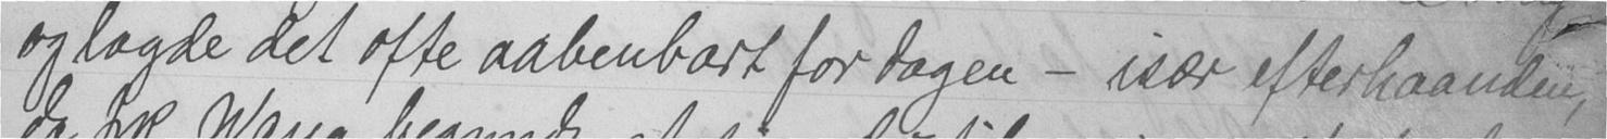og lagde det ofte aabenbart for dagen - især efterhaanden,
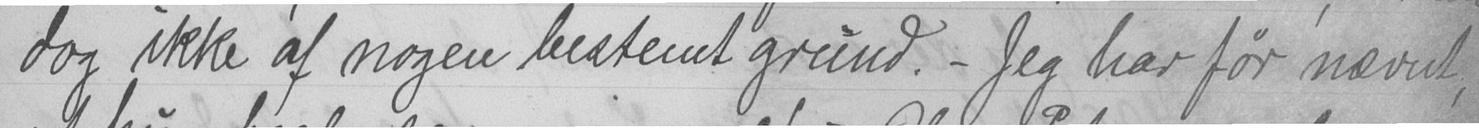dog ikke af nogen bestemt grund. - Jeg har før nævnt,
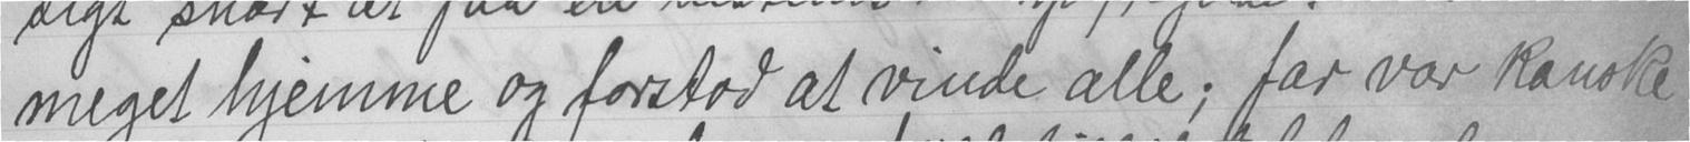meget hjemme og forstod at vinde alle; far var kanske
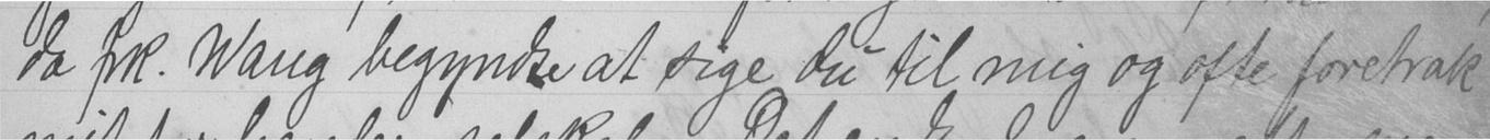da frk. Wang begyndte at sige du til mig og ofte foretrak
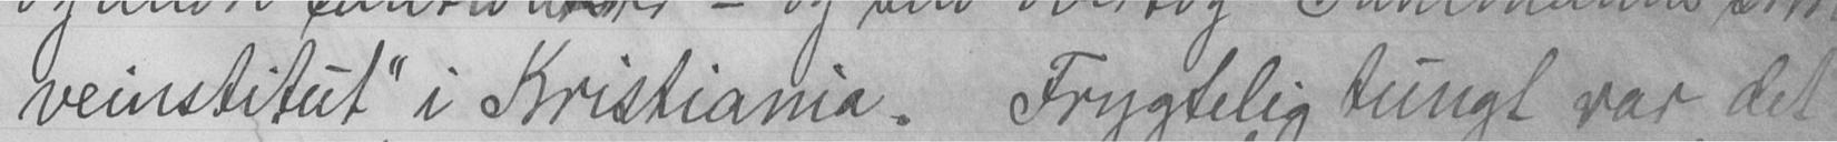veinstitut" i Kristiania. Frygtelig tungt var det
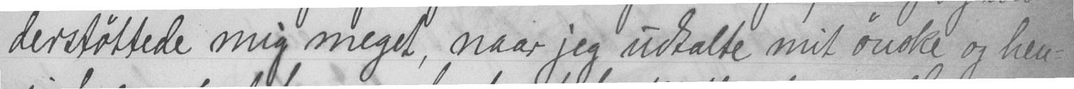derstøttede mig meget, naar jeg udtalte mit ønske og hen-
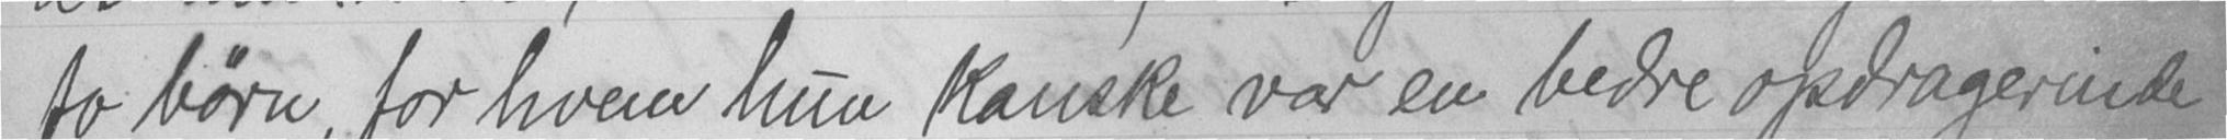to børn, for hvem hun kanske var en bedre opdragerinde
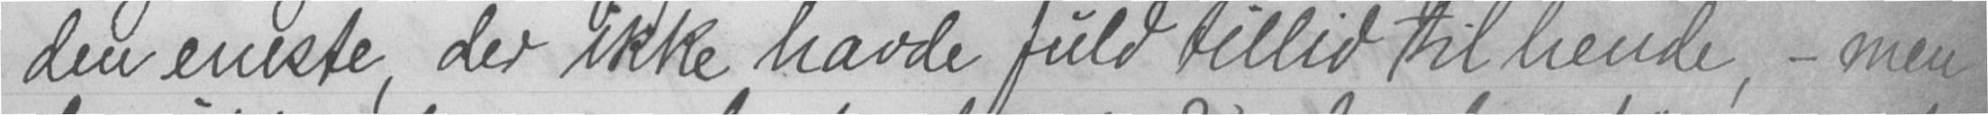den eneste, der ikke havde fuld tillid til hende, - men
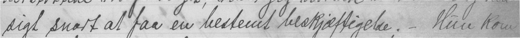sigt snart at faa en bestemt beskjæftigelse. - Hun kom
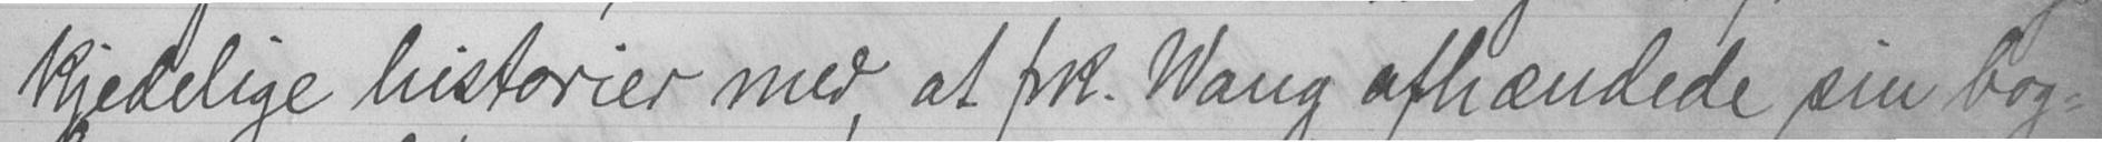kjedelige historier med, at frk. Wang afhændede sin bog-
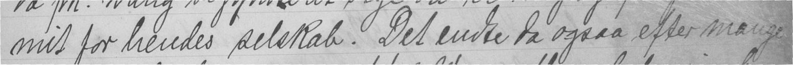mit for hendes selskab. Det endte da ogsaa efter mange
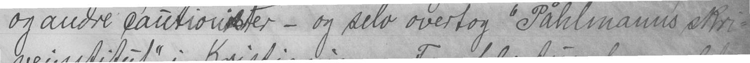og andre cautionister - og selv overtog "Påhlmanns skri-
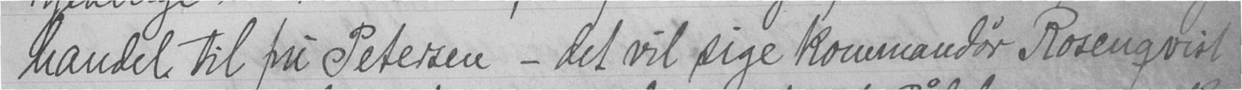handel til fru Petersen - det vil sige kommandør Rosenqvist
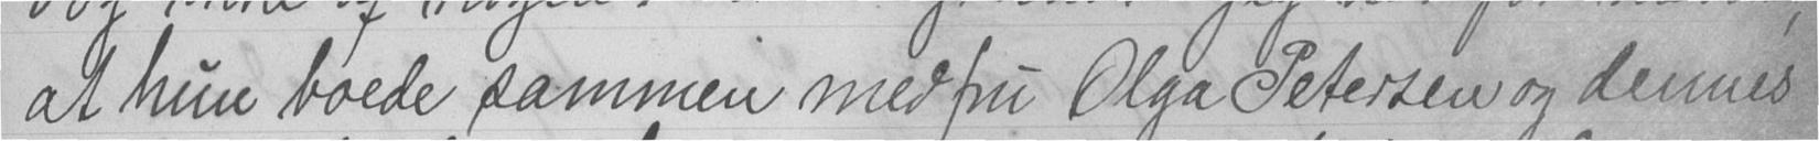at hun boede sammen med fru Olga Petersen og dennes
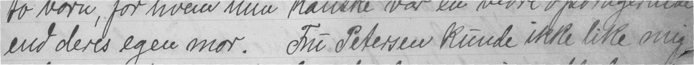end deres egen mor. Fru Petersen kunde ikke like mig
